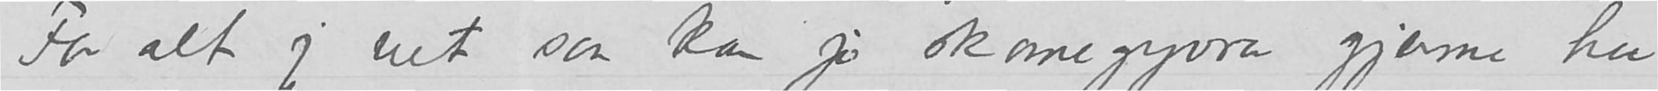For alt jeg vet saa kan jo skarregyvren gjerne hu
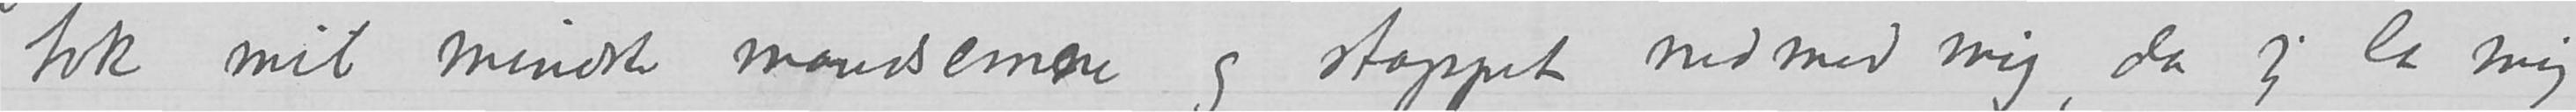tok mit mindste mandsemne og stappet ned med mig, da jeg la mig
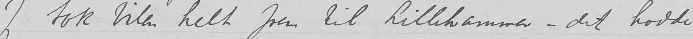Jeg tok bilen helt frem til Lillehammer – det hadde
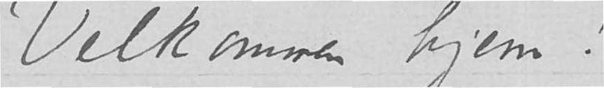Velkommen hjem!
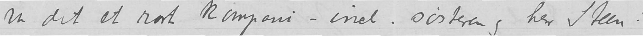var det et rart kompani – incl. søsteren og herr Steen!
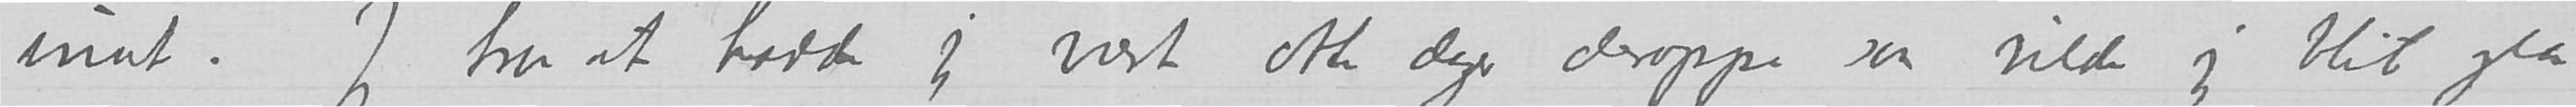inat. Jeg tror at hadde jeg vært otte dager deroppe saa vilde jeg blit gla
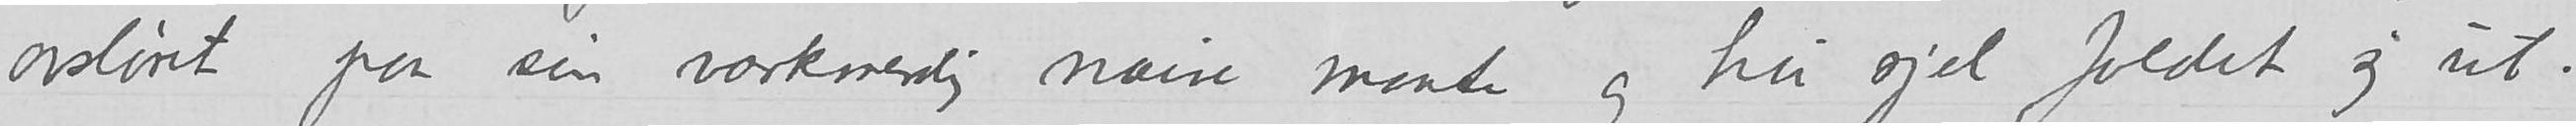avsløret paa sin mærkverdig naive maate og hu sjel foldet sieg ut.
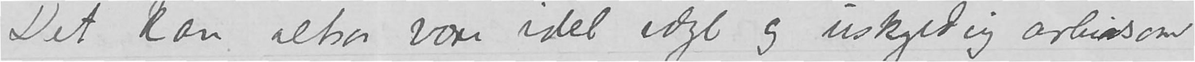Det kan altsaa være idel idyl og uskyldig arbeidsom
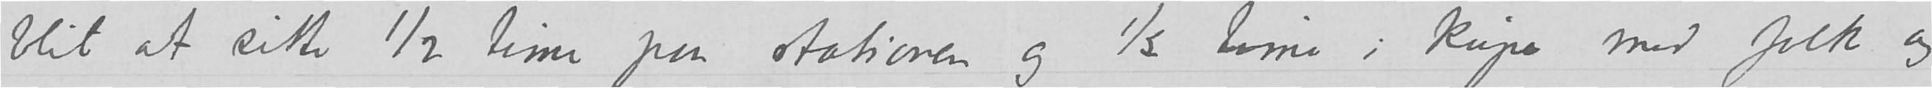blit at sitte ½ time paa stationenog <½> time i kupe med folk og
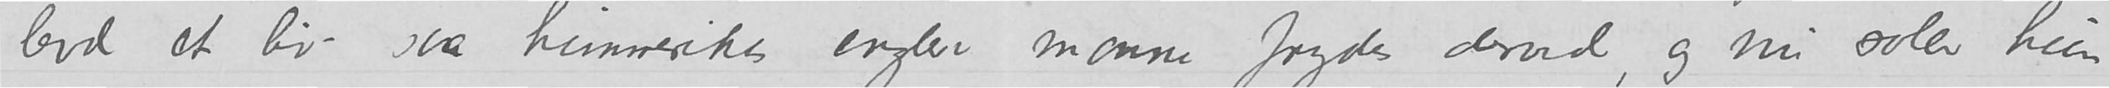levd et liv saa himmerikes engler monne frydes derved, og nu
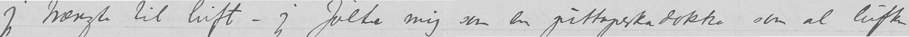jeg trængte til luft – jeg følte mig som en puittopeskadokke som al luften
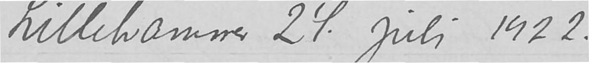Lillehammer 24. juli 1922.
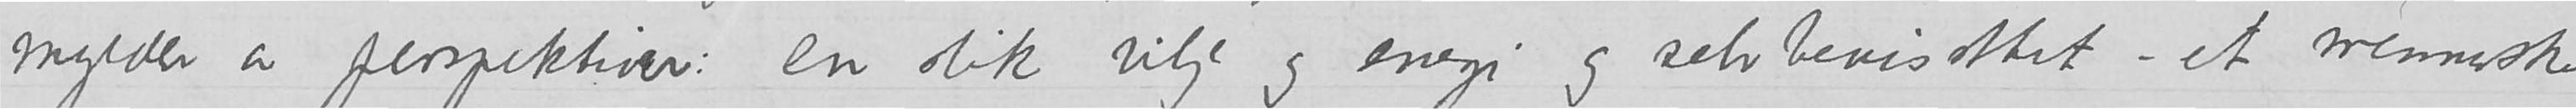mylder av perspektiver: en slik vilje og energi og selvbevissthet – et menneske
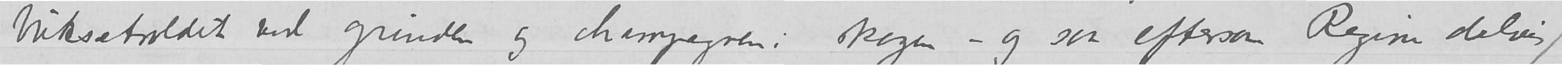buksetroldet ved grinden og champagnen i skogen – og saa eftersom Regine delvis
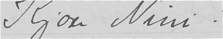Kjære Nini
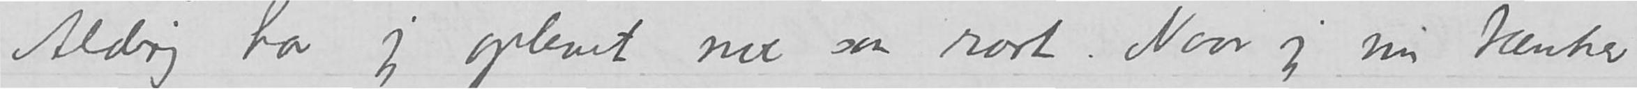Aldrig har jeg oplevet noe saa rart. Naar jeg nu tænker
